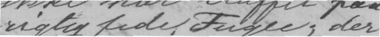rigtig fede Fugle; der
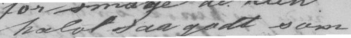halvt saa godt som
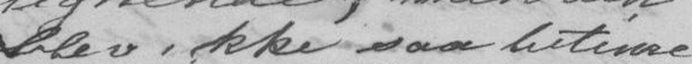blev ikke saa betime
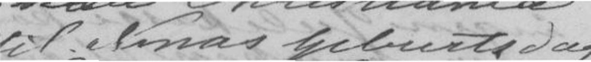til Ninas Geburtsdag.
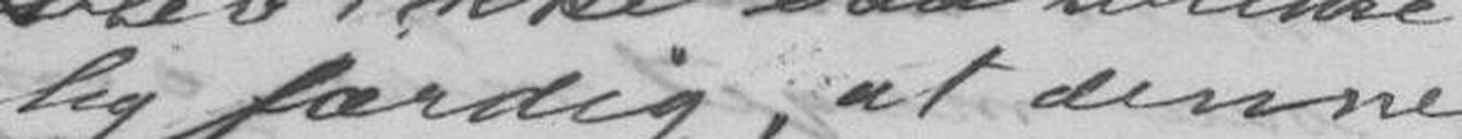lig færdig, at denne
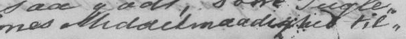nes Middelmaadighed til
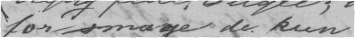for smage de kun
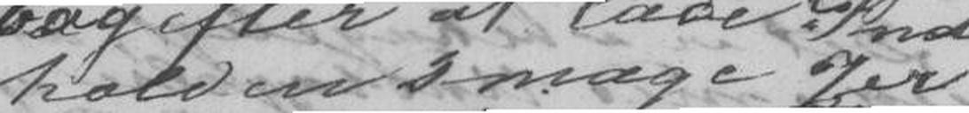holden smage Jer
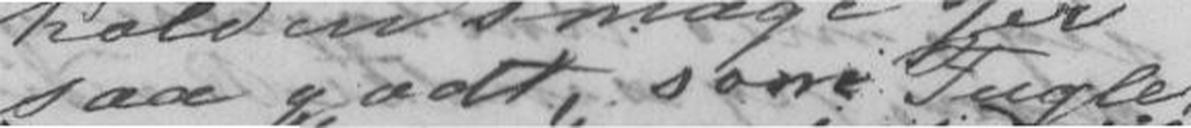saa godt, som Fugle-
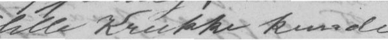lille Krukke kunde
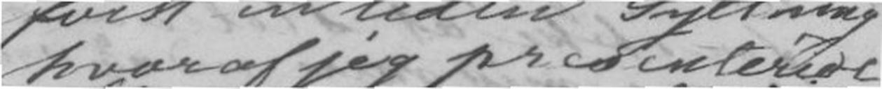hvoraf jeg presenterede
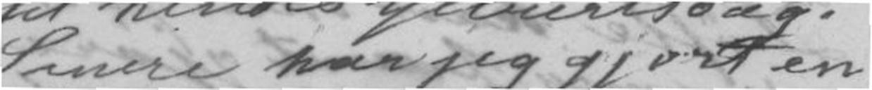Senere har jeg gjort en
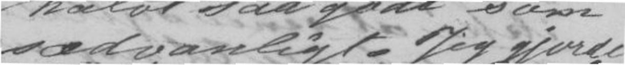sædvanligt. Jeg gjorde
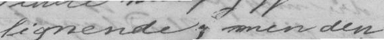lignende; men den
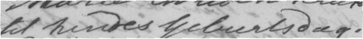til hendes Geburtsdag.
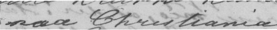naa Christiania
